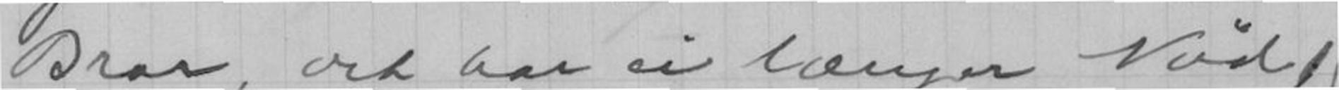Bror, det har ei længre Nød!
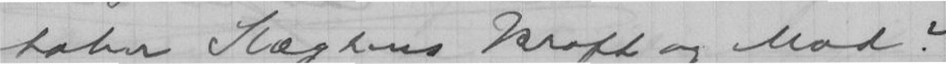taber Slægtens Kraft og Mod?
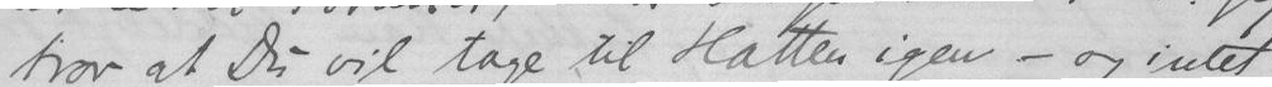tror at Du vil tage til Hatten igen - og intet
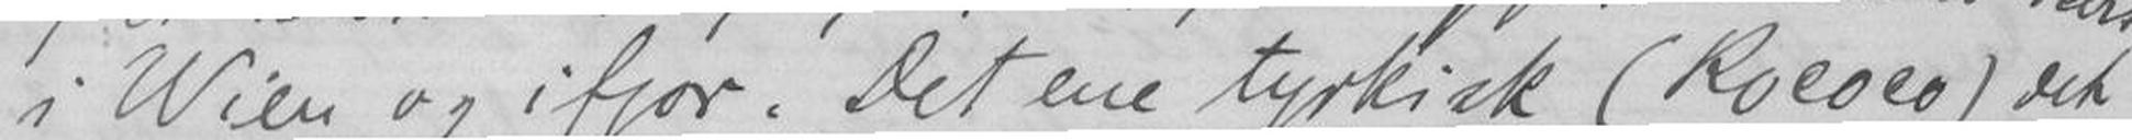i Wien og i fjor. Det ene tyrkisk (Rococo) det
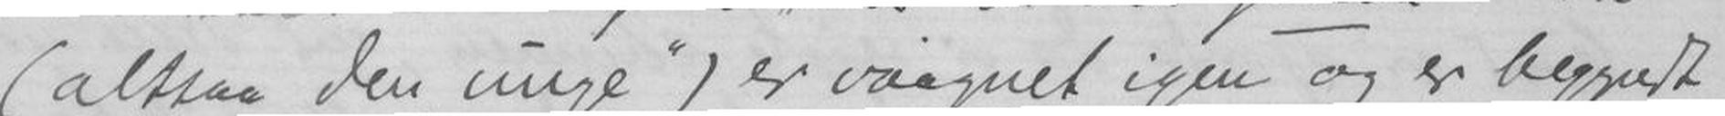(altsaa den "unge") er vaagnet igen og er begyndt
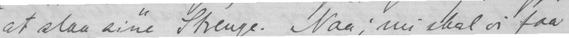at slaa sine Strenge. Naa; nu skal vi faa
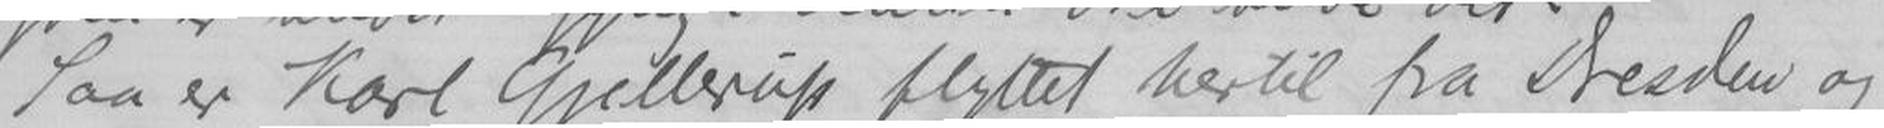Saa er Karl Gjellsrup flyttet hertil fra Dresden og
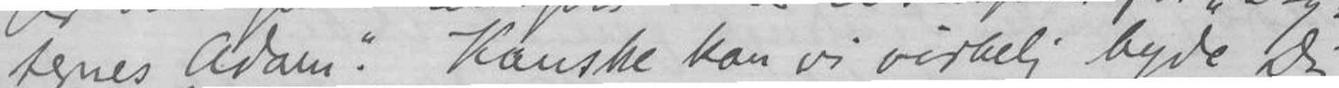ternes Adam". Kanske kan vi virkelig byde Dig
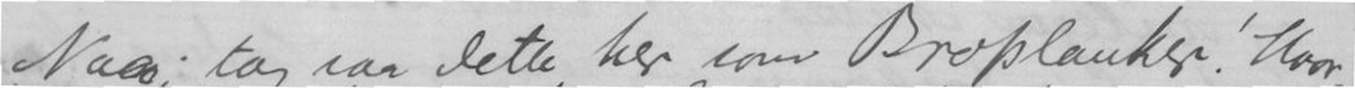Naa; tag saa dette her som Broplanker! Hvor
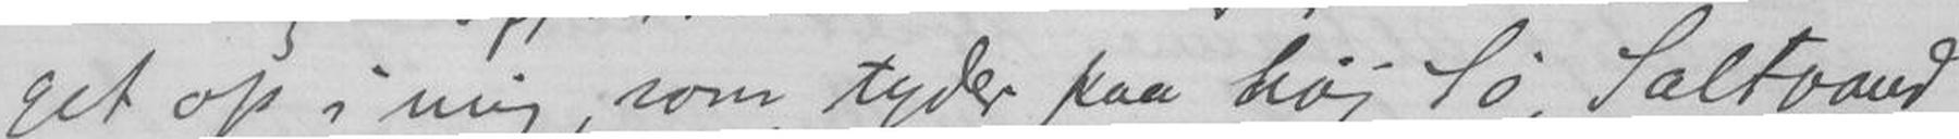get op i mig, som tyder paa høj Sø, Saltvand
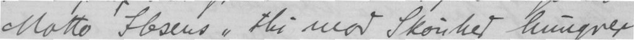Motto Ibsen "thi mod Skønhed hungrer
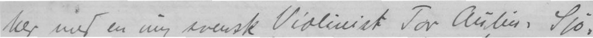ker med en svensk Violinist Tor Aulin, Sjø-
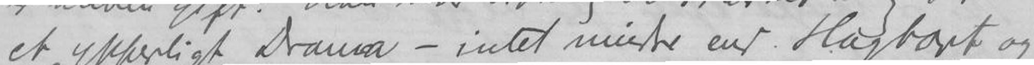et ypperligt Drama - intet mindre end Hagbart og
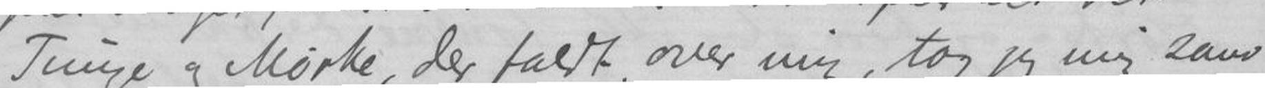Tunge og Mørke, der faldt over mig, tog jeg mig sam-
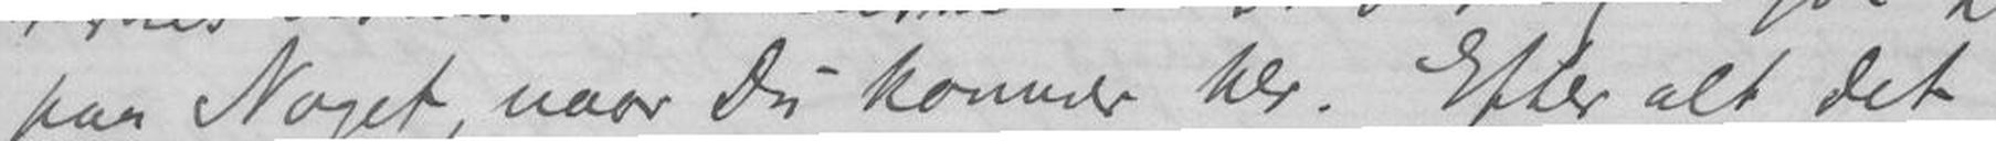paa Noget, naar Du kommer her. Efter alt det
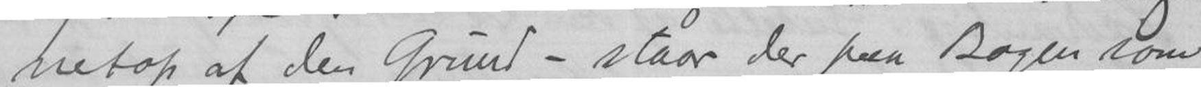netop af den Grund - Staar der paa Bogen som
